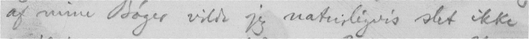af mine Bøger vilde jeg naturligvis slet ikke
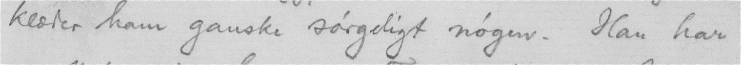klæder ham ganske sørgeligt nøgen. Han har
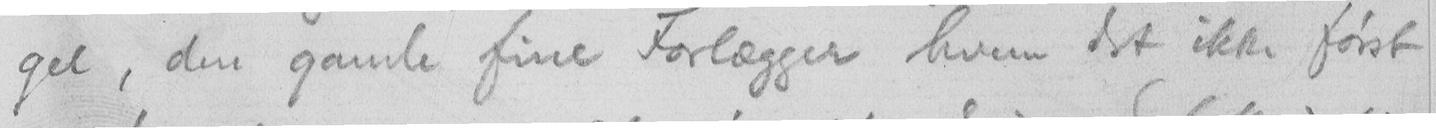gel, den gamle fine Forlægger hvem det ikke først
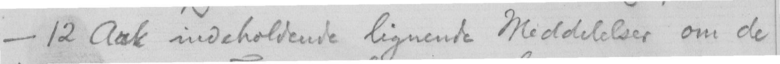-12 Ark indeholdende lignende Meddelelser om de
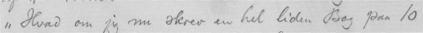"Hvad om jeg nu skrev en hel liden Bog paa 10
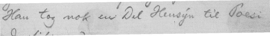Han tog nok en Del Hensyn til Poesi
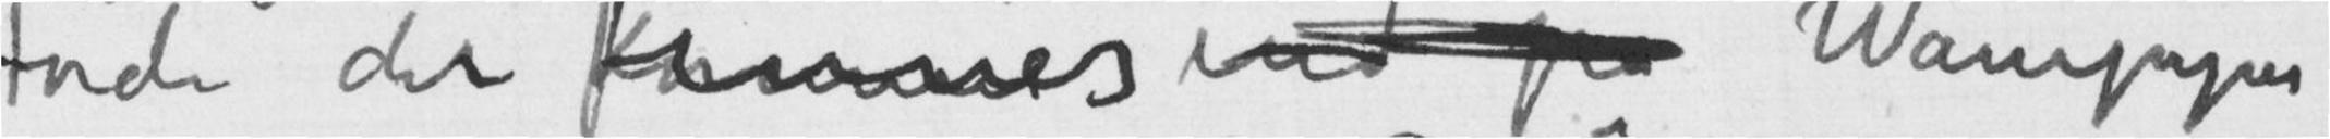fordi der finnes end på Wampyrer
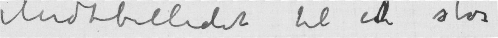Midtbilledet til ent stor
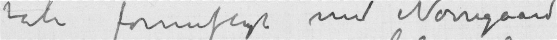tale fornuftigt med Nørregaard
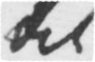det
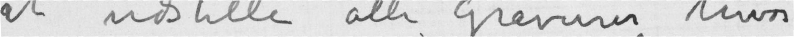at udstille alle Gravurer hvis
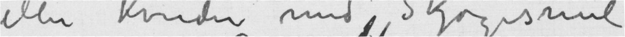eller Kvinden med Skjøgesmil
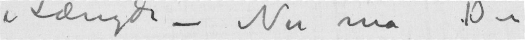i Længde – Nu må Du
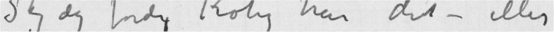Skjæg fordi Krohg har det – eller
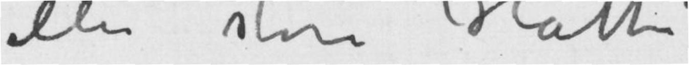eller store Hatte
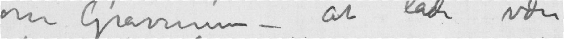om Gravurerne – At lade være
